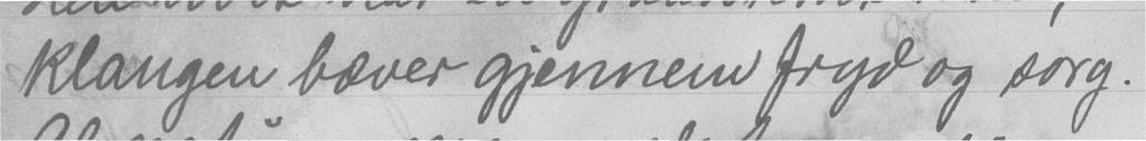klangen bæver gjennem fryd og sorg.
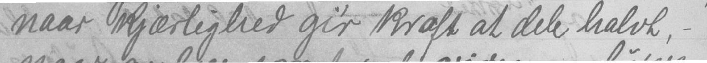naar kjærlighed gir kraft at dele halvt, -
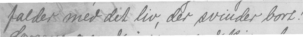falder med det liv, der svinder bort!
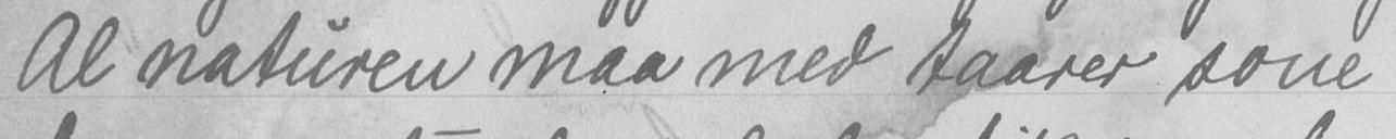Al naturen maa med taarer sone
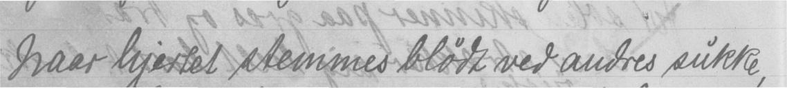Naar hjertet stemmes blødt ved andres sukke,
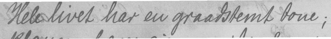Hele livet har en graadstemt tone;
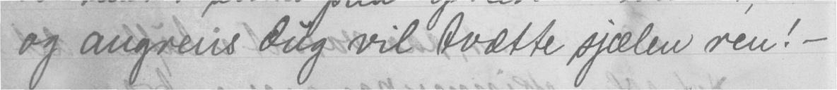og angrens dug vil tvætte sjælen ren! -
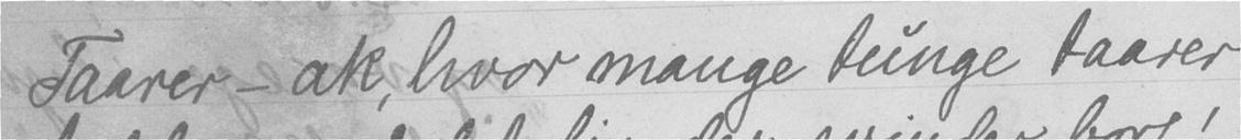Taarer - ak, hvor mange tunge taarer
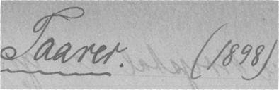Taarer. (1898)
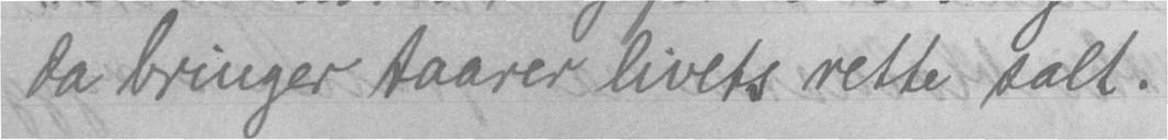da bringer taarer livets rette salt.
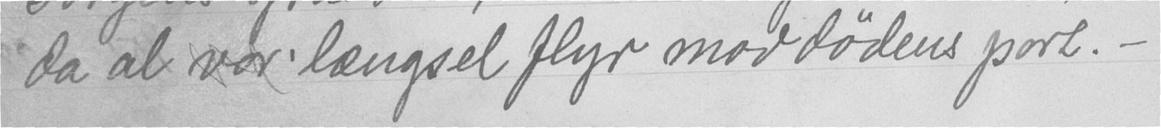da al vor længsel flyr mod dødens port. -
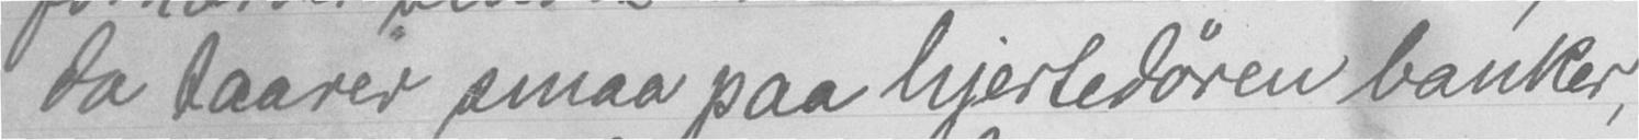da taarer smaa paa hjertedøren banker,
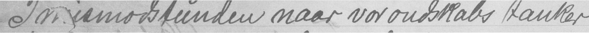I mismodstunden naar vor ondskabs tanker
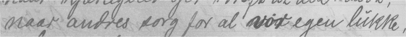naar andres sorg for al vor egen lukke,
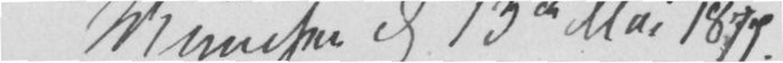München den 13de Mai 1877.
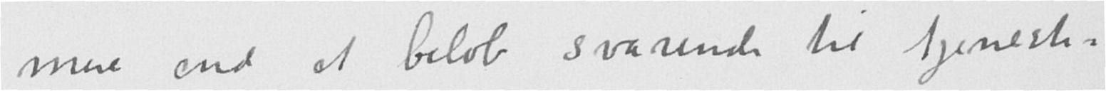mer end et beløb svarende til tjeneste-
